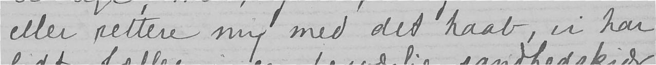eller rettere mig med det haab, vi har
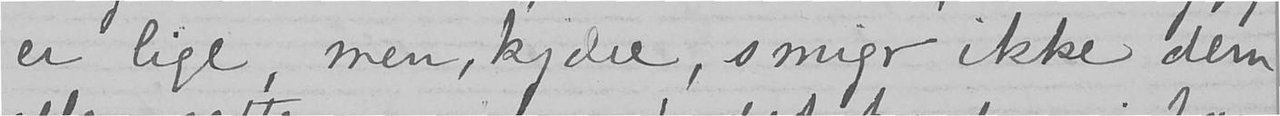er lige, men, kjære, smigr ikke dem
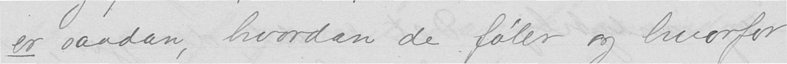er saadan, hvordan de føler og hvorfor
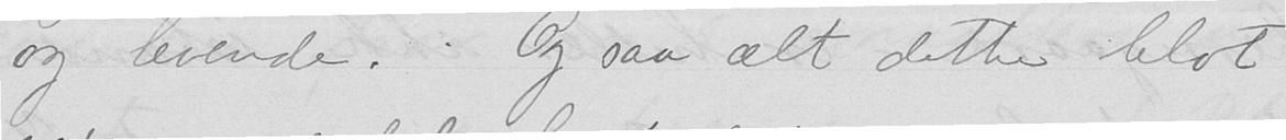og levende. Og saa alt dette blot
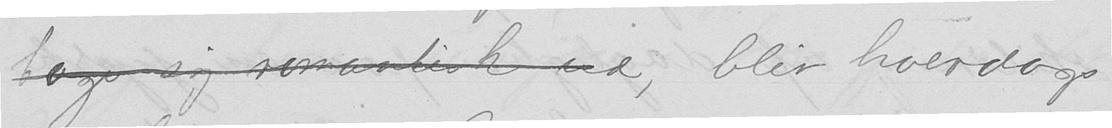tage sig romantisk ud, blir hverdags
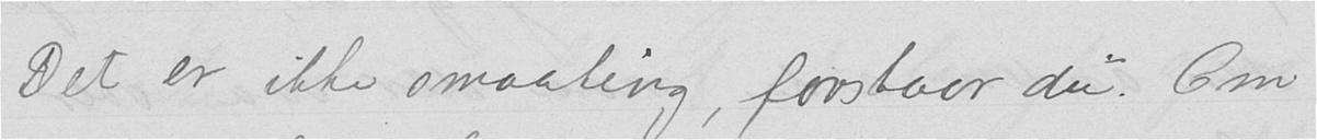Det er ikke smaating, forstaar du. Om
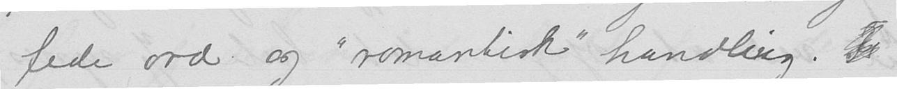fede ord og "romantisk" handling.
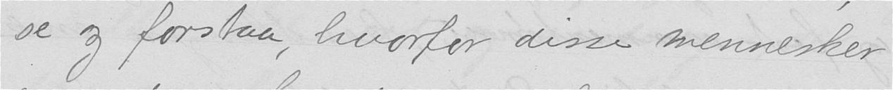se og forstaa, hvorfor disse mennesker
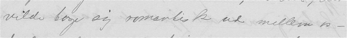vilde tage sig romantisk ud mellem os -
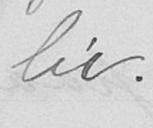liv.
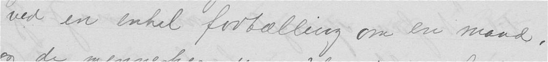ved en enkel fortælling om en mand,
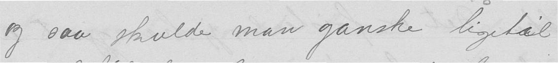og saa skulde man ganske ligetil
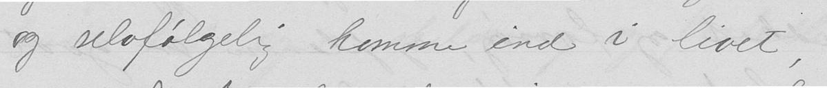og selvfølgelig komme ind i livet,
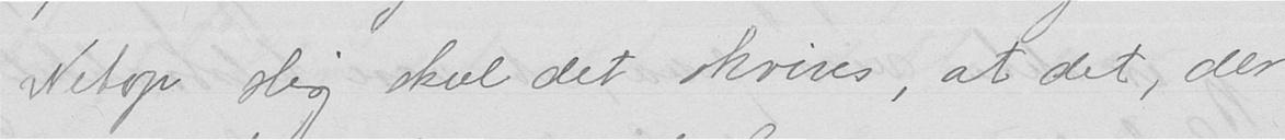Netop slig skal det skrives, at det, der
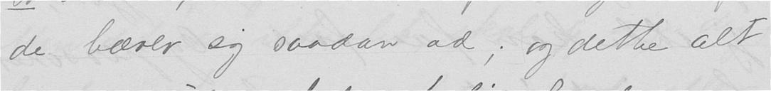de bærer sig saadan ad; og dette alt
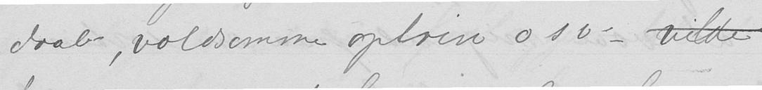drab, voldsomme optrin osv. vilde
